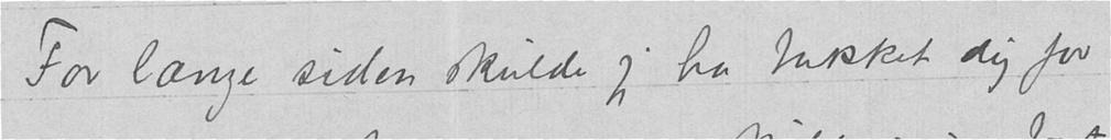For længe siden skulde jeg ha takket dig for
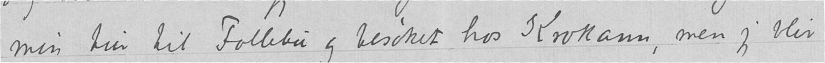min tur til Follebu og besøket hos Krokann, men jeg blir
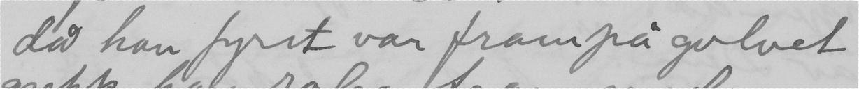då han fyrst var frampå golvet
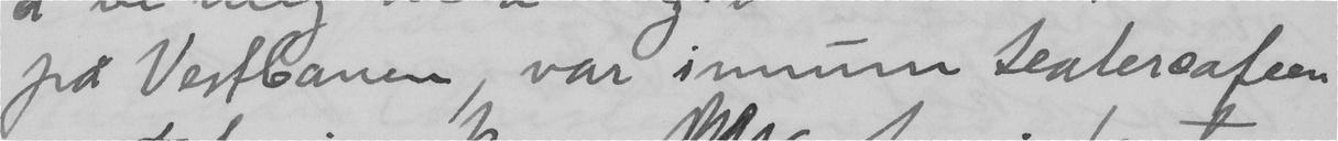på Vestbanen, var innum Teatercafeen
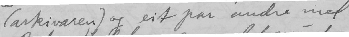(arkivaren) og eit par andre med
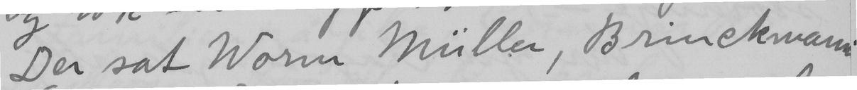Der sat Worm Müller, Brinckmann
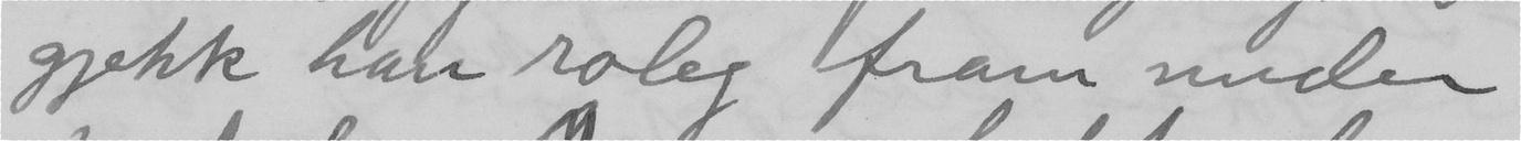gjekk han roleg fram under
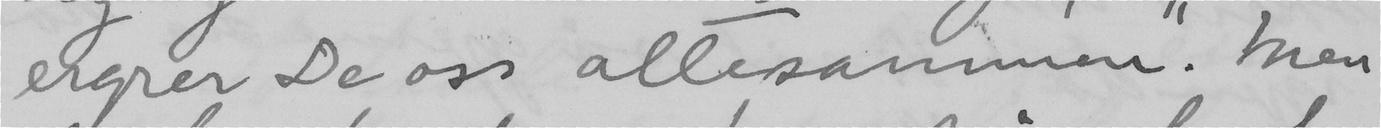ergrer De oss allesammen". Men
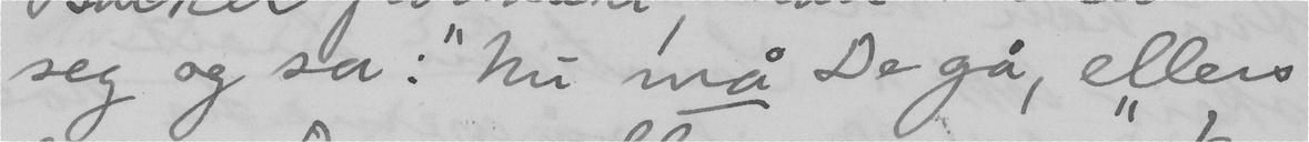seg og sa: "Nu må De gå, ellers
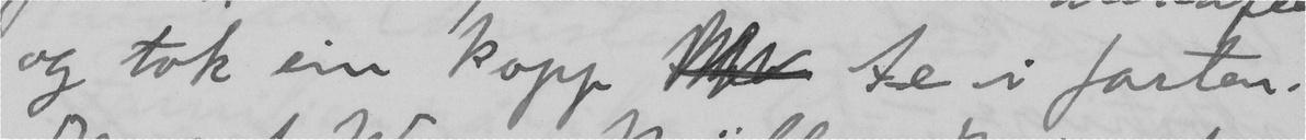og tok ein kopp  te i farten.
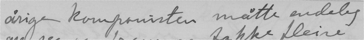årige komponisten måtte endeleg
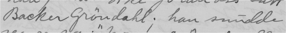Backer Gröndahl; han snudde
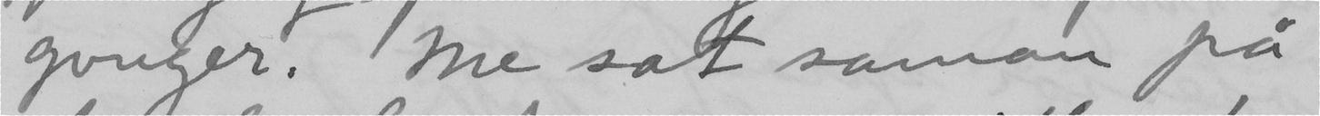gonger. Me sat saman på
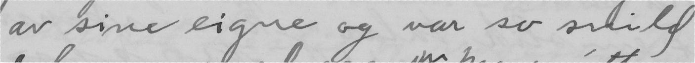av sine eigne og var so snild
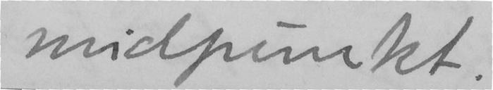midpunkt.
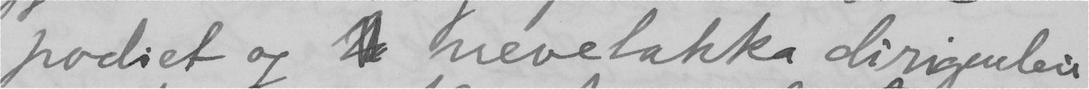podiet og  nevetakka dirigenten
